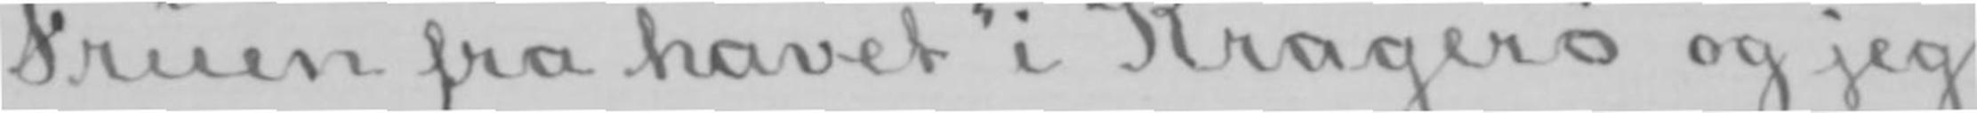«Fruen fra havet» i Kragerø og jeg
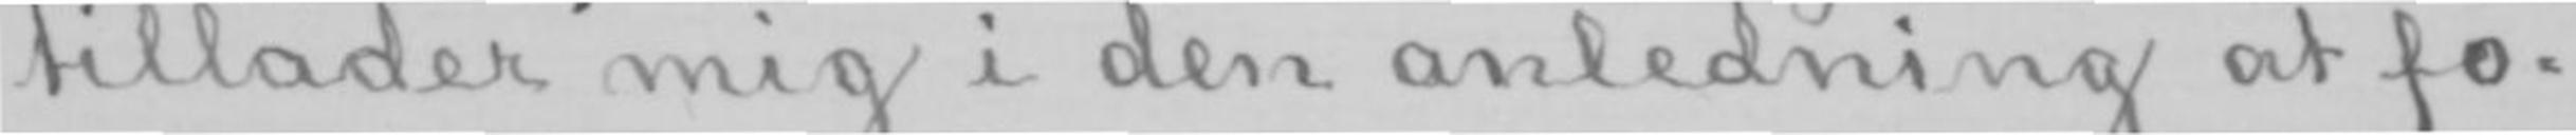tillader mig i den anledning at fo-
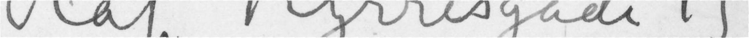Olaf Kyrresgade 11
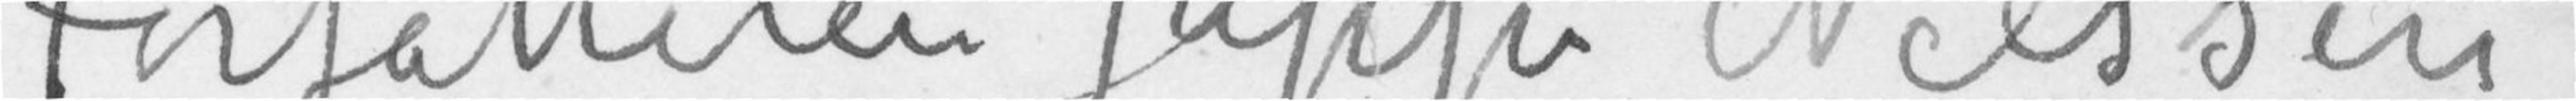Forfatteren Jappe Nilssen
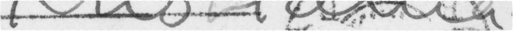Kristiania
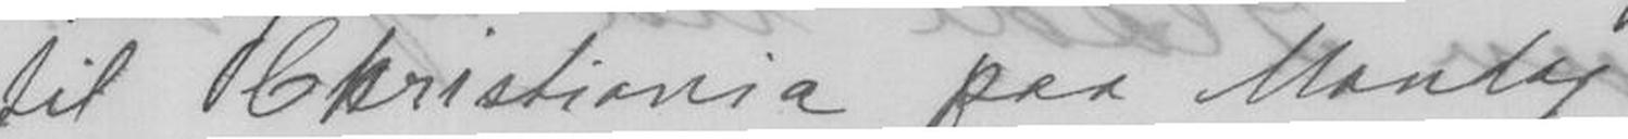til Kristiania paa Mandag.
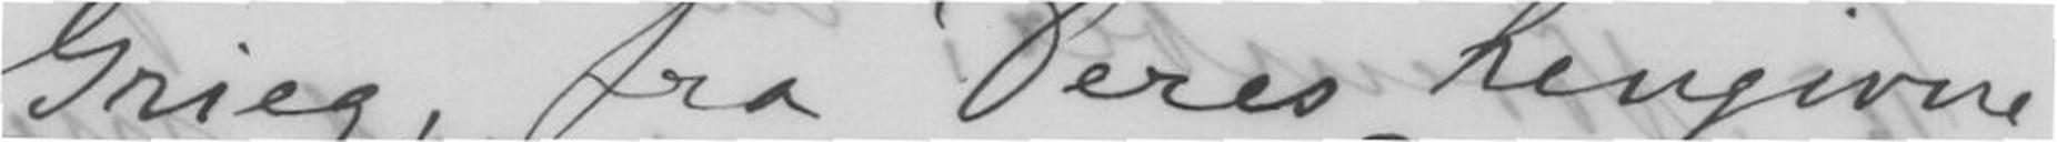Grieg, fra Deres hengivne
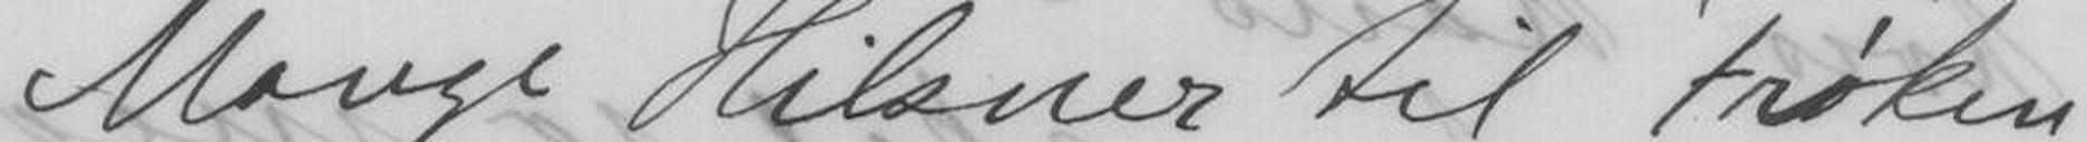Mange Hilsner til Frøken
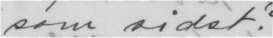som sidst?
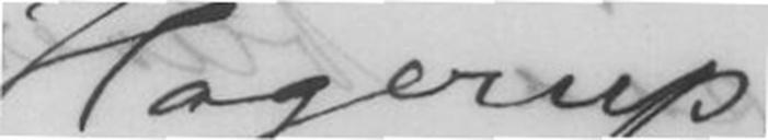Hagerup
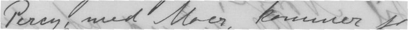Percy, med Moer, kommer jo
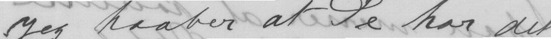Jeg haaber at De har det
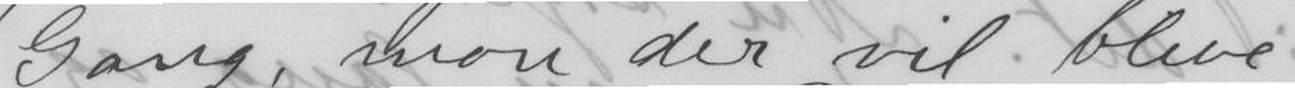Gang, mon der vil blive
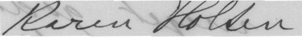Karen Holten
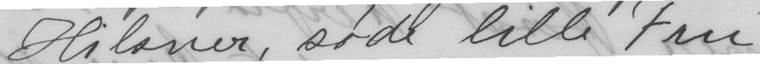Hilsner, søde lille Fru
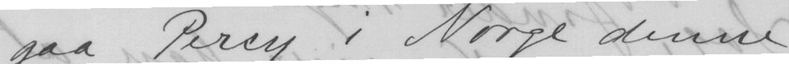gaa Percy i Norge denne
